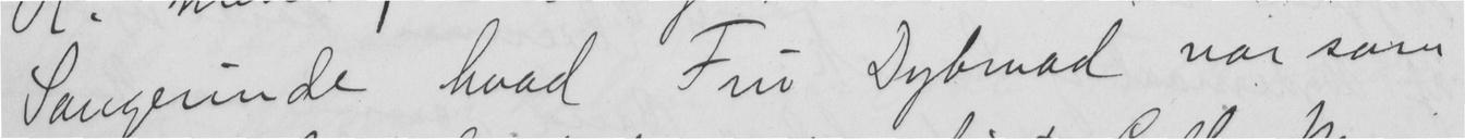Sangerinde hvad Fru Dybwad var som
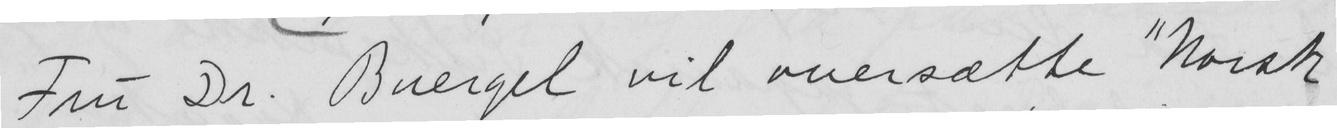Fru Dr. Buergel vil oversætte "Norsk
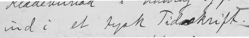ind i et tysk Tidsskrift
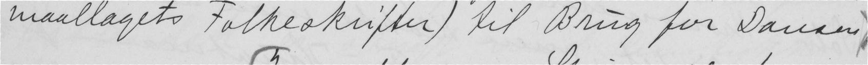maallagets Folkeskrifter) til Brug for Dansere
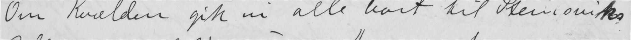Om Kvælden gik vi alle bort til Steinsviks
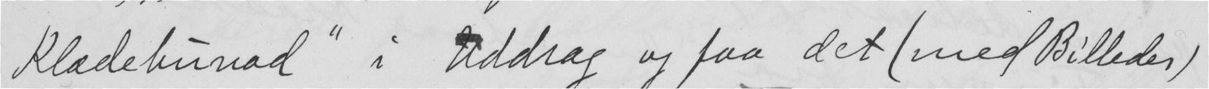Klædebunad" i Uddrag og faa det (med Billeder)
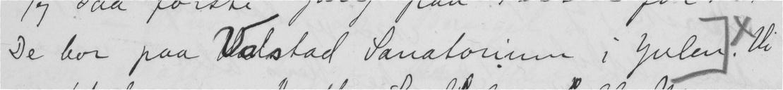De bor paa Valstad Sanatorium i Julen. Vi
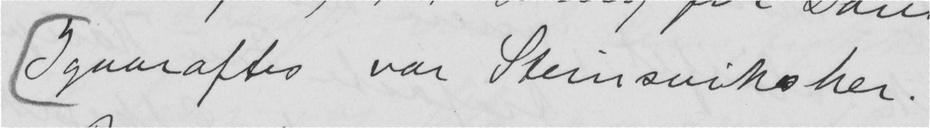Igaaraftes var Steinsviks her.
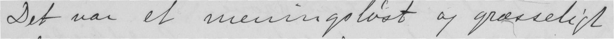Det var et meningsløst og græsseligt
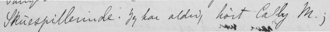Skuespillerinde. Jeg har aldrig hørt Cally M.;
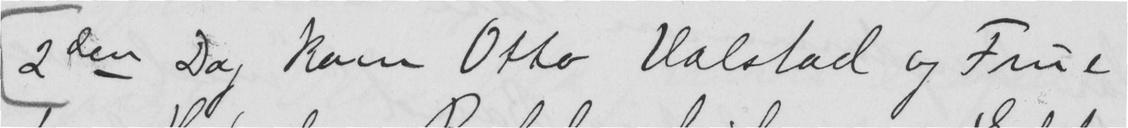2den Dag kom Otto Valstad og Frue
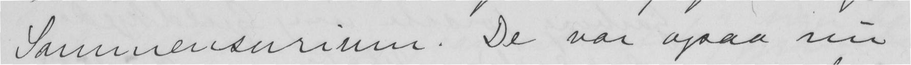Sammensurium. De var ogsaa nu
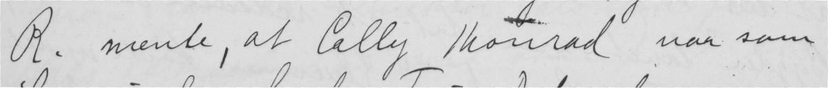R. mente, at Cally Monrad var som
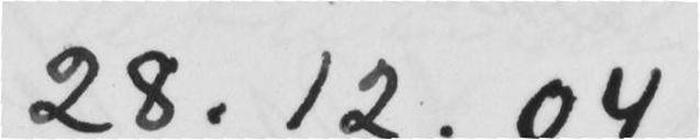28.12.04
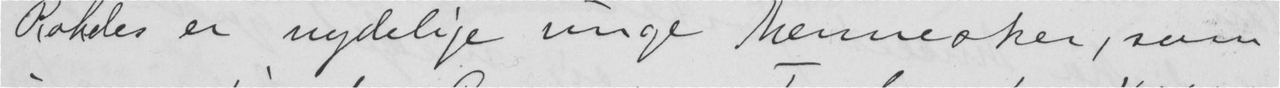Rohdes er nydelige unge Mennesker, som
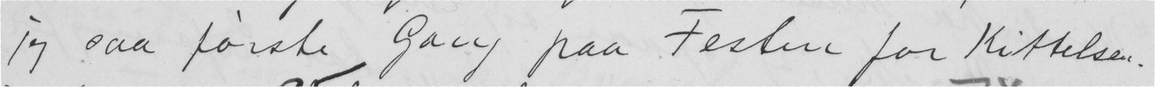jeg saa første Gang paa Festen for Kittelsen.
